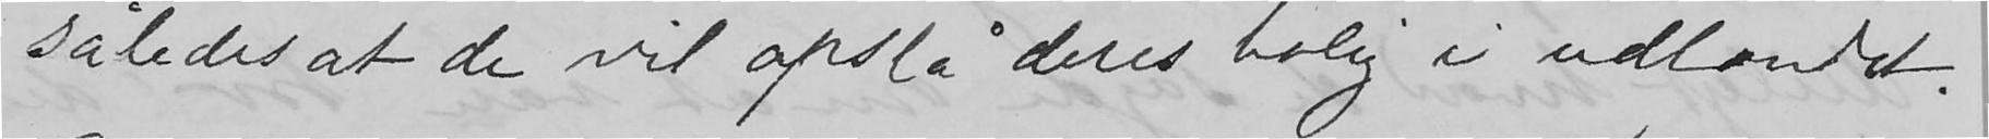således at de vil opslå deres bolig i udlandet.
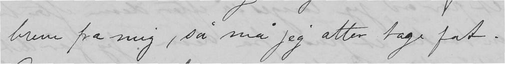breve fra mig, så må jeg atter tage fat.
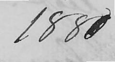1880
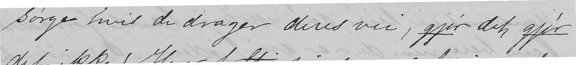sørge hvis de drager deres vei, gjør det, gjør
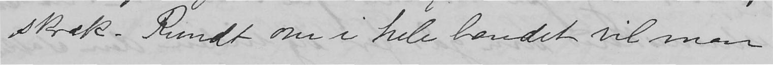skræk. Rundt om i hele landet vil man
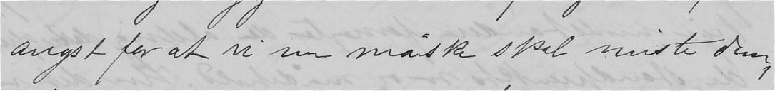angst for at vi nu måske skal miste dem,
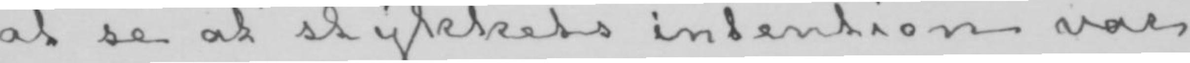at se at stykkets intention var
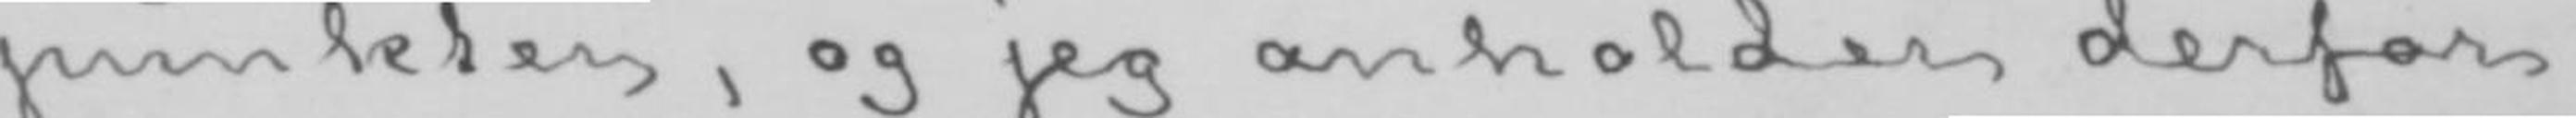punkter, og jeg anholder derfor
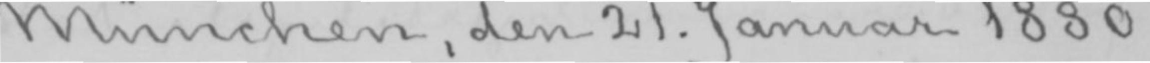München, den 21. Januar 1880.
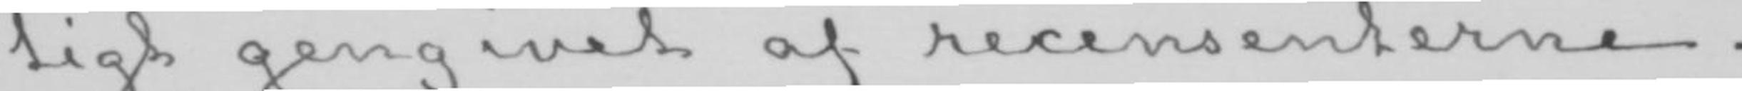tigt gengivet af recensenterne.
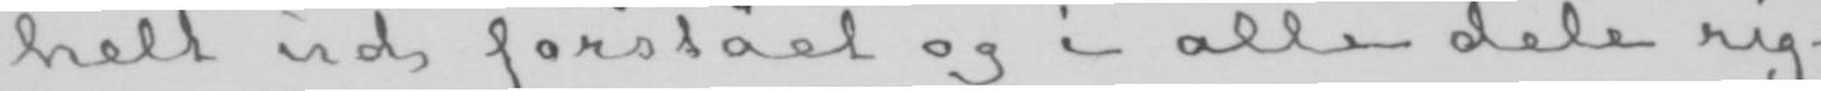helt ud forstået og i alle dele rig-
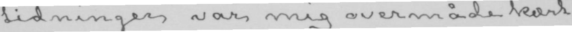tidninger var mig overmåde kært
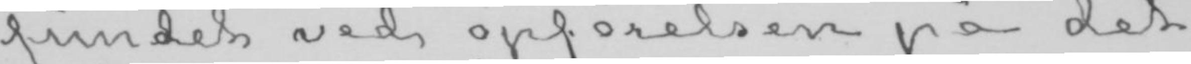fundet ved opførelsen på det
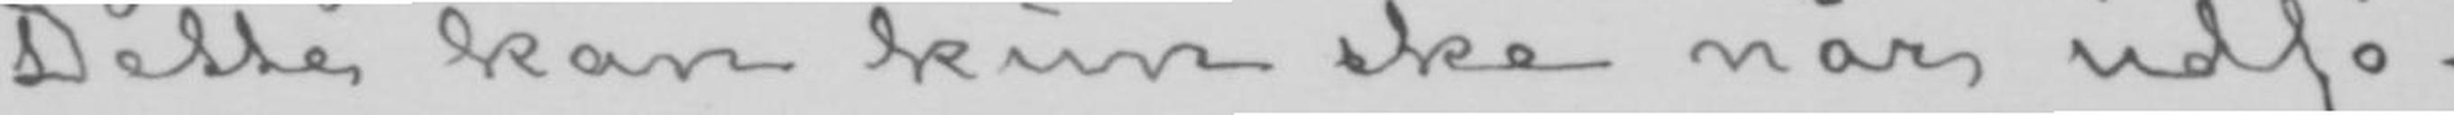Dette kan kun ske når udfø-
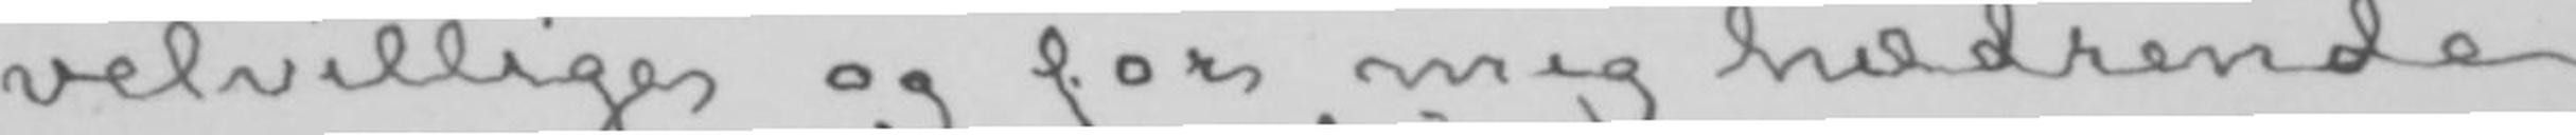velvillige og for mig hædrende
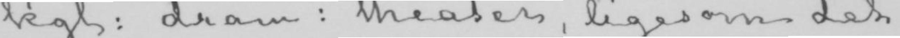kgl: dram: theater, ligesom det
